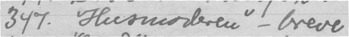347. Husmoderen" - breve
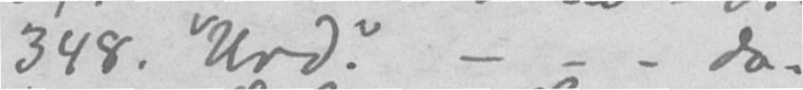348. "Urd." - - - da.
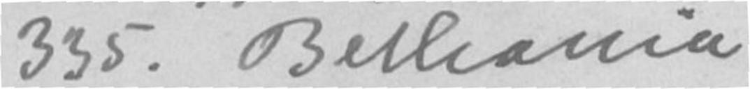335. Belhania
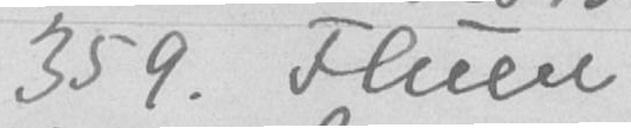359. Fluen
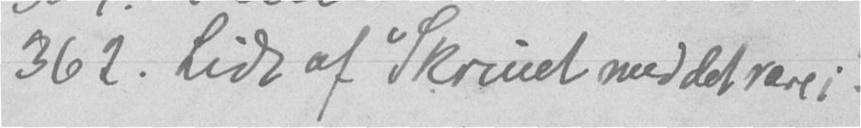362. Lidt af "Skrinet med det rare i".
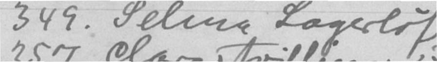349. Selma Lagerløf
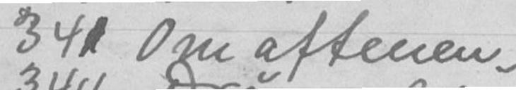341. Om aftenen.
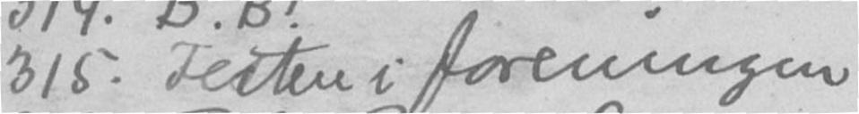315. Festen i foreningen
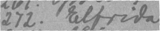272. Elfrida
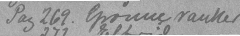Pag 269. Grønne ranker
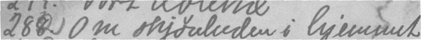282. Om skjønheden i hjemmet
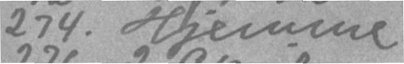274. Hjemme
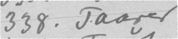338. Taarer
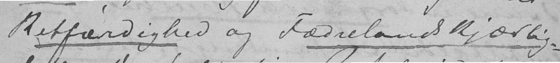Retfærdighed og Fædrelandskjærlig-
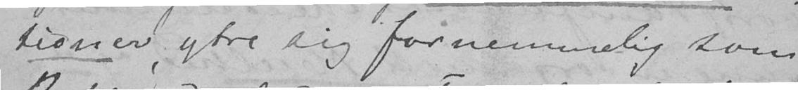tioner, ytre sig fornemmelig som
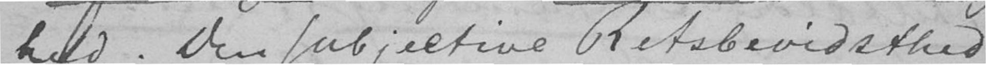hed. Den subjective Retsbevidsthed
